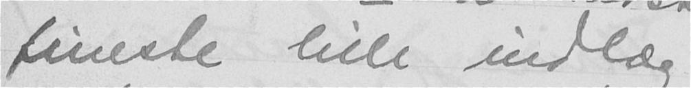fineste lille indlæg
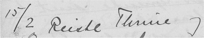15/2 Reiste Thrine og
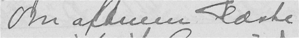Om aftenen læste
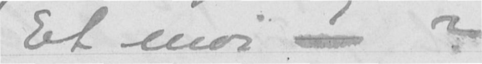Et moi - ?
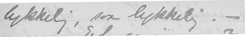lykkelig, saa lykkelig. -
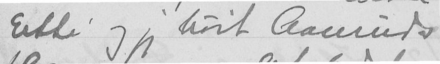Etti og jeg høit Aanruds
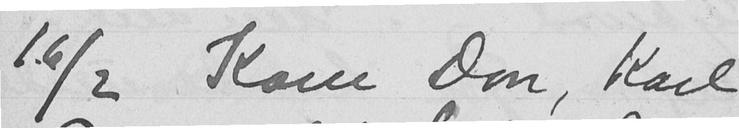16/2 Kom Don, Karl
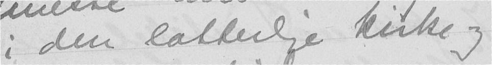i den latterlige kirke og
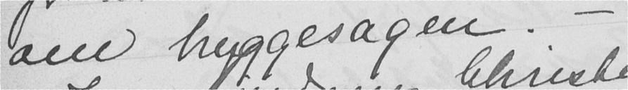om bryggesagen -
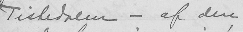Tistedalen - af den
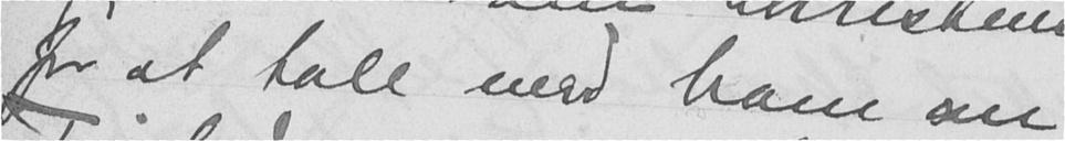for at tale med ham om
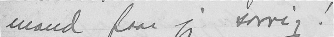mand faar jeg sorrig!
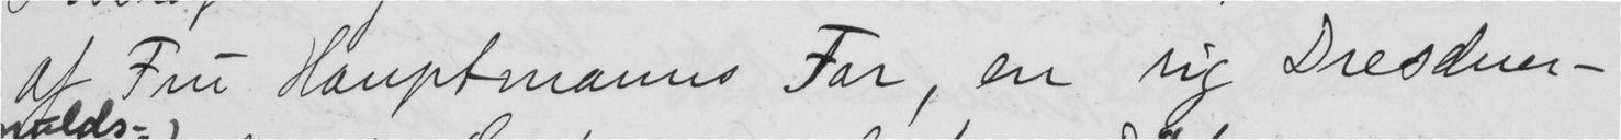af Fru Hauptmanns Far, en rig Dresdner -
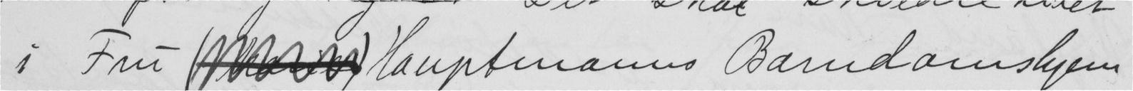i Fru  Hauptmanns Barndomshjem
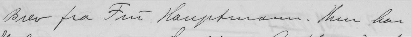Brev fra Fru Hauptmann. Hun har
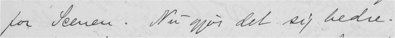for Scenen. Nu gjør det sig bedre.
